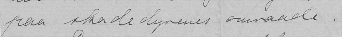paa skadedyrenes omraade.
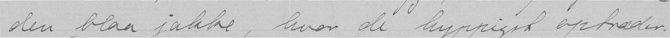den blaa jakke, hvor de hyppigst optræder.
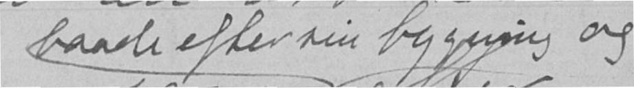baade efter sin bygning og
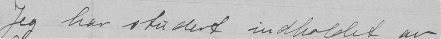Jeg har studere indholdet av
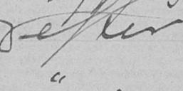efter
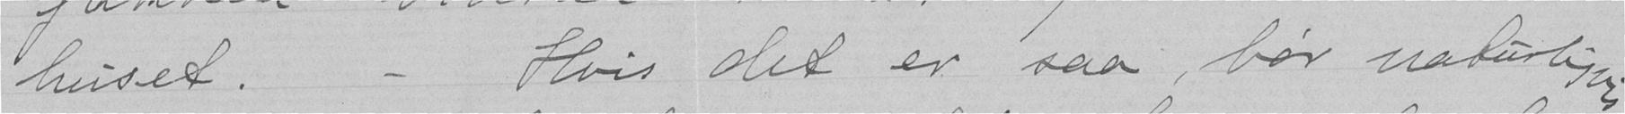huset. - Hvis det er saa, bør naturligvis
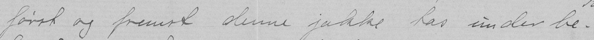først og fremst denne jakke has under be-
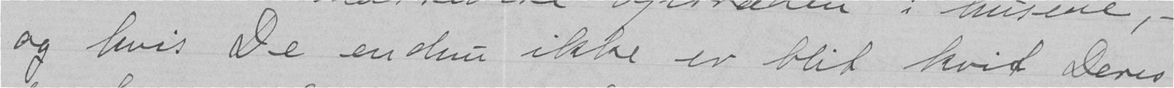og hvis De endnu ikke er blit kvit Deres
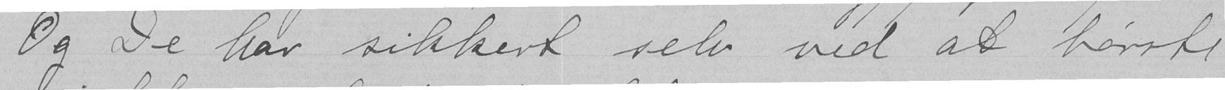Og De har sikkert selv ved at børste
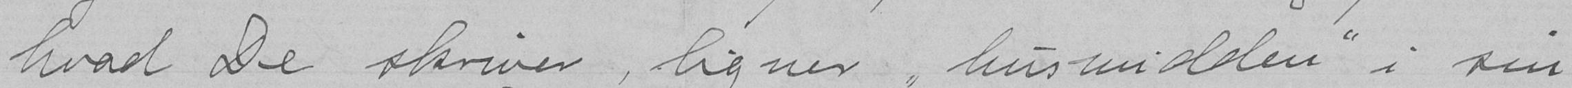hvad De skriver, ligner husmidden i sin
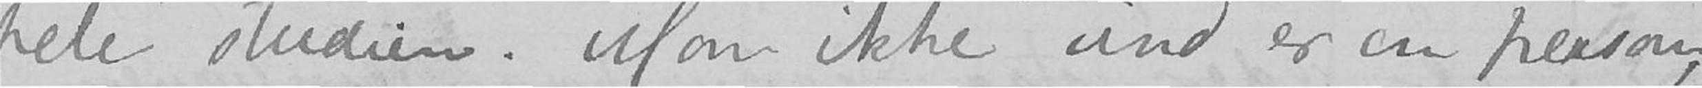hele studien. Mon ikke vind er en person,
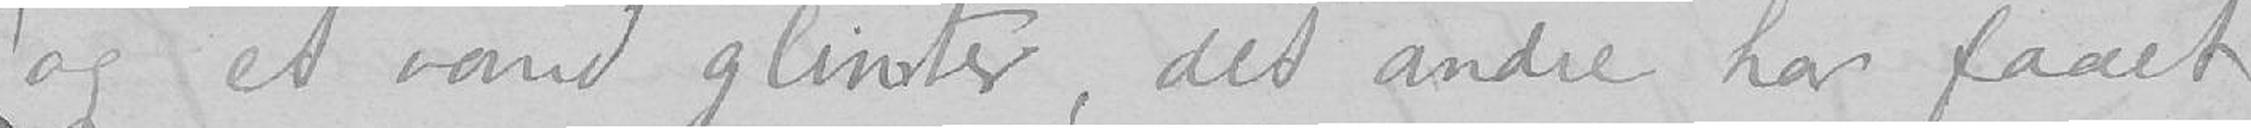og et vand glimter, det andre har faaet
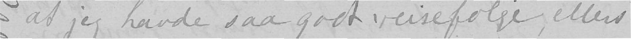at jeg havde saa godt reisefølge, ellers
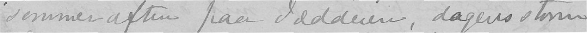sommeraften paa Jædderen, dagens storm
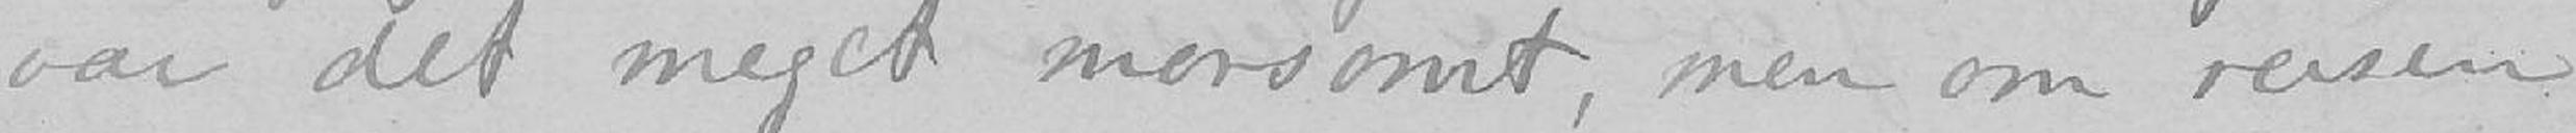var det meget morsomt, men om reisen
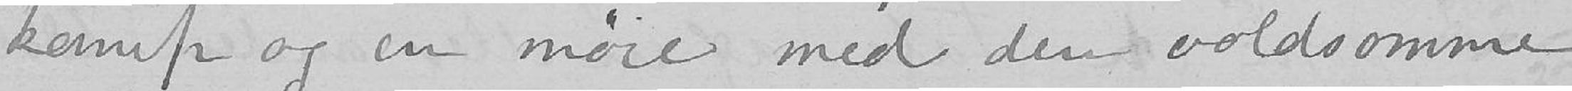kamp og en møie med den voldsomme
